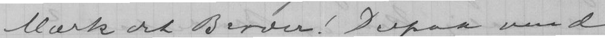Mærk det Broder! Derpaa vend
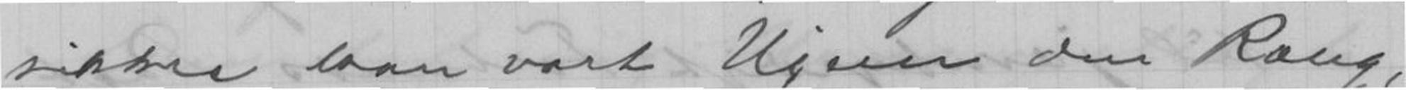sikker kan vort Hjem den Rang,
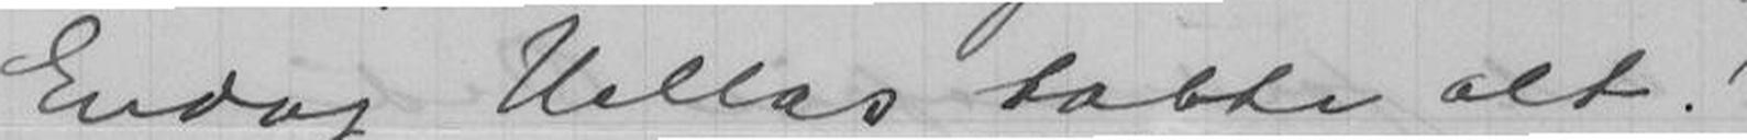Endog Hellas tabte alt!
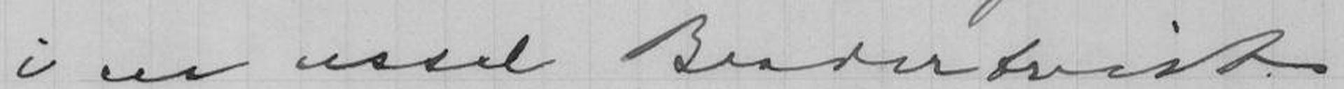i en ussel Brodertvist
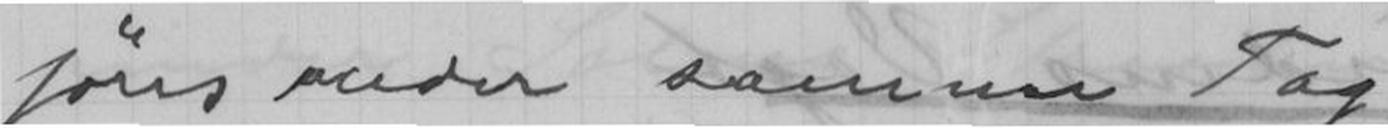føres under samme Tag,
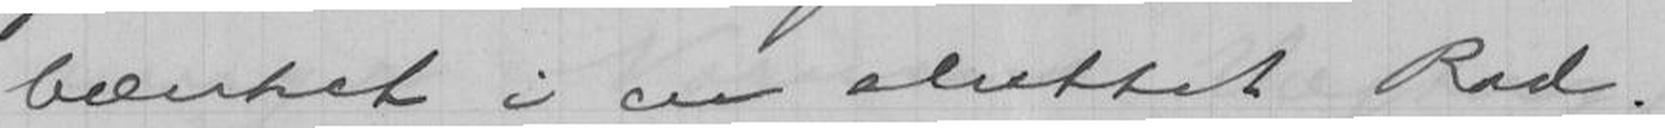bænket i en sluttet Rad!
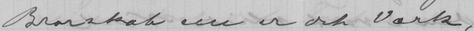Brorskab nu er det Værk,
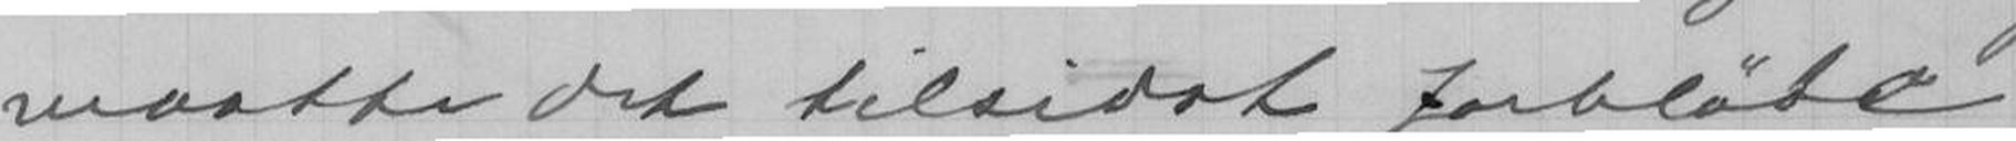maatte det tilsidst forbløde
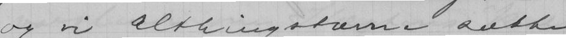og vi Althingstævne sætter
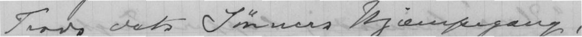Trods dets Sønners Kjæmpegang,
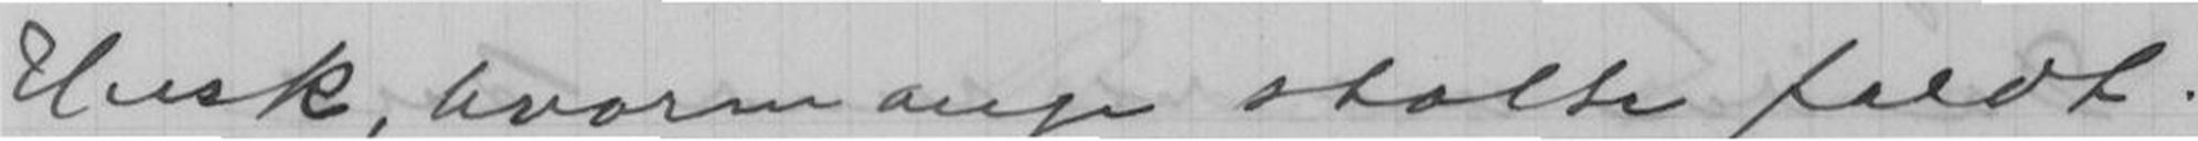Husk, hvormange stolte faldt!
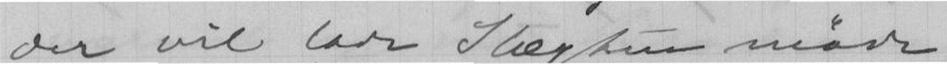der vil lade Slægten møde
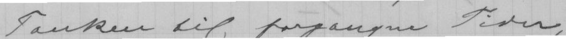Tanken til forgangne Tider,
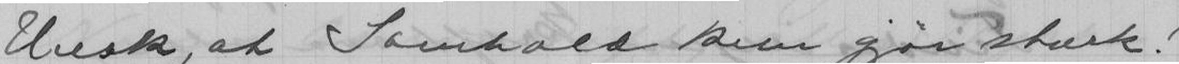Husk, at Samhold kun gjør stærk!
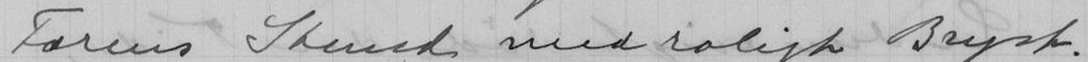Farens Stund med roligt Bryst.
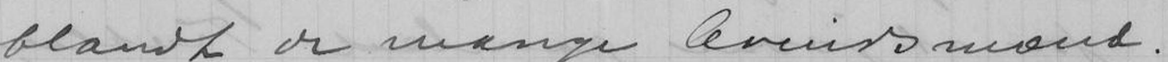blandt de mange Ærindsmænd.
